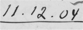11.12.04
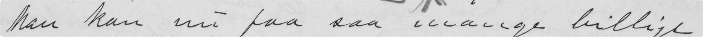Man kan nu faa saa mange billige
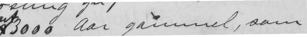3000 Aar gammel, som
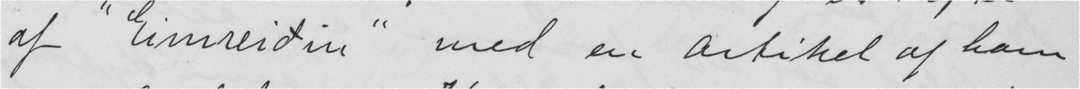af "Eimreidin" med en Artikel af ham
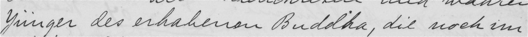Yünger des erhabenen Buddha, die noch im
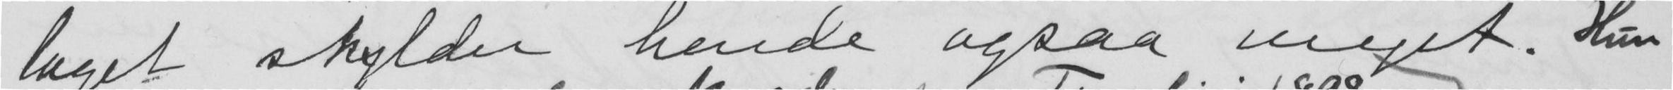laget skylder hende ogsaa meget. Hun
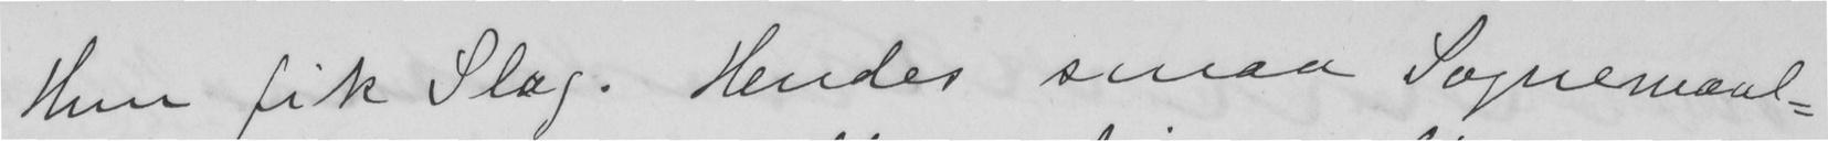Hun fik Slag. Hendes smaa Sognemaal-
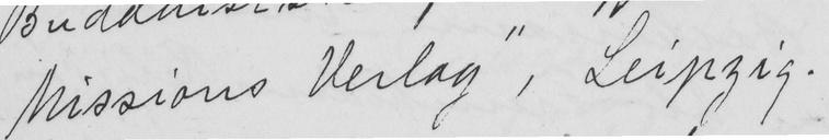Missions Verlag. Leipzig.
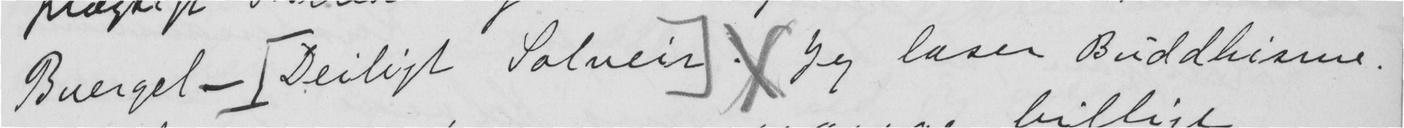Buergel - Deiligt Solveir. Jeg læser Buddhisme.
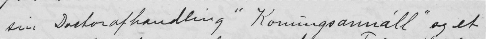sin Doctorafhandling "Konungsannáll" og et
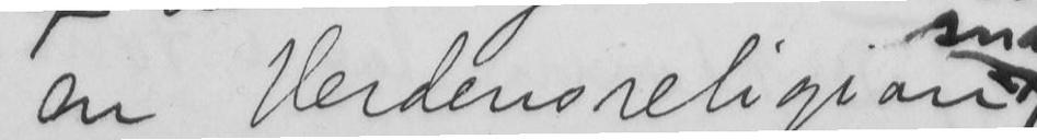en Verdensreligion
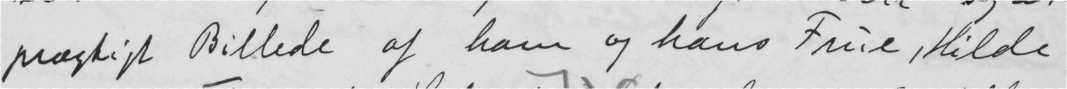prægtigt Billede af ham og hans Frue, Hilde
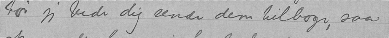tør jeg bede dig sende dem tilbage, saa
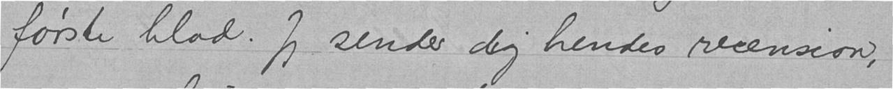første blad. Jeg sender dig hendes recension,
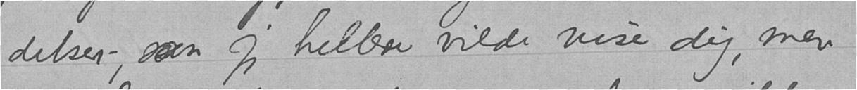elser -, som jeg heller vilde vise dig, men
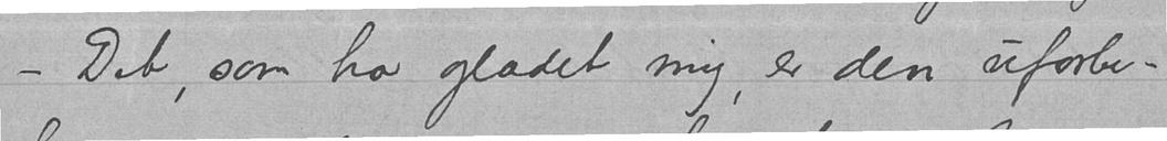- Det, som har glædet mig, er den uforbe-
